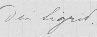Din Sigrid.
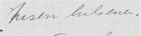tusen hilsener,
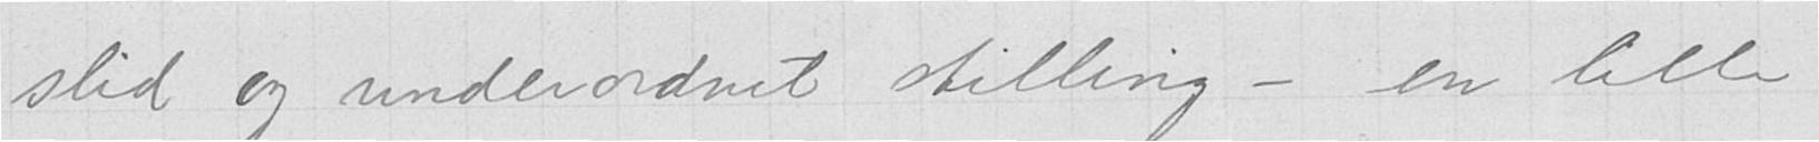slid og underordnet stilling – en lille
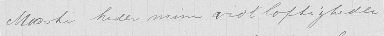Maaske keder mine vidtløftigheder
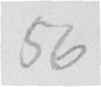56
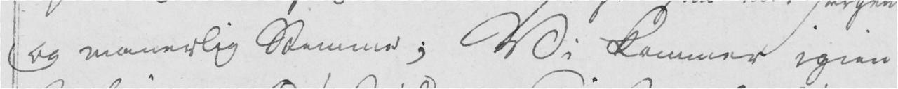og mannerlig Stemme; Vi kommer igien
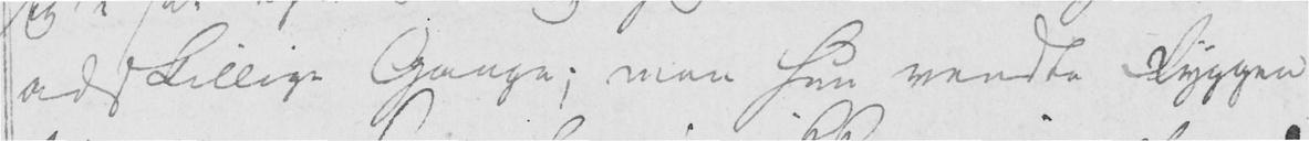adskillige Gange; men hun vendte Ryggen
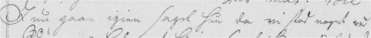I nu gaae igien sagde hun da vi stod noget ved
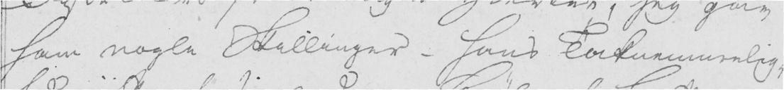ham nogle skillinger - Hans Taknemmelig-
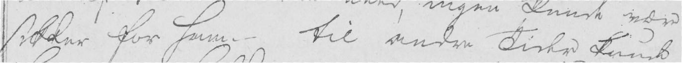sikker for ham - til andre Tider kunde
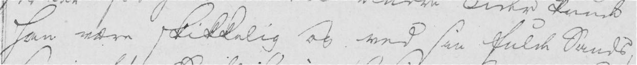han være skikkelig og ved sin fulde Sands,
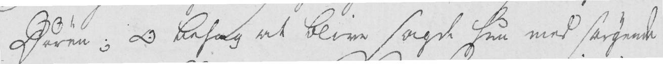Døren; O behag at blive sagde hun med sørgende
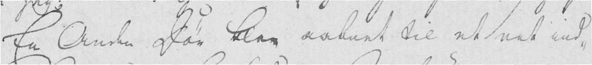En Anden Dør blev aabnet til et ret ind-
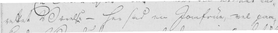rettet Værelse - her sad en Jomfrue, vel paa-
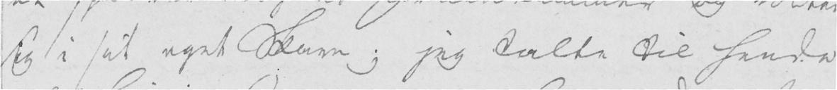sig i sit eget Skarn; jeg talte til hende
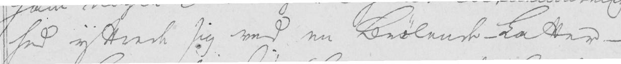hed ytrede sig ved en Brølende-Latter -
